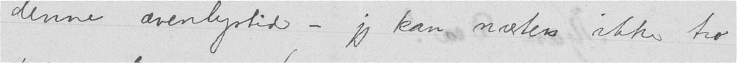denne æventyrtid - jeg kan næsten ikke tro
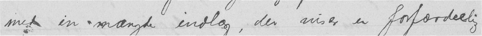med en mængde indlæg, der viser en forfærdelig
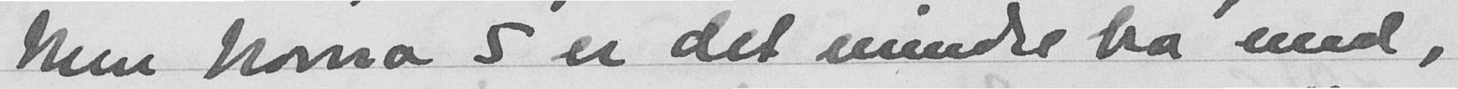Men Norna 5 er det mindre bra med,
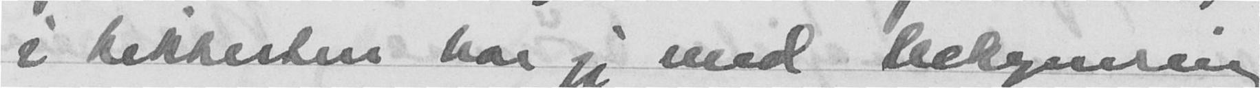i kikkerten har jeg med bekymring
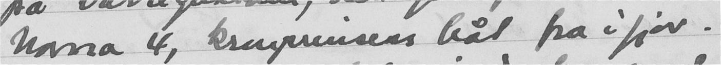Norna 4, kronprinsens båt fra i fjor.
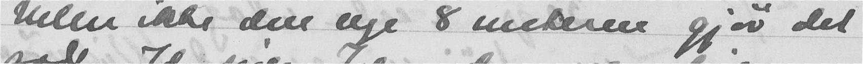heller ikke den nye 8 meteren gjør det
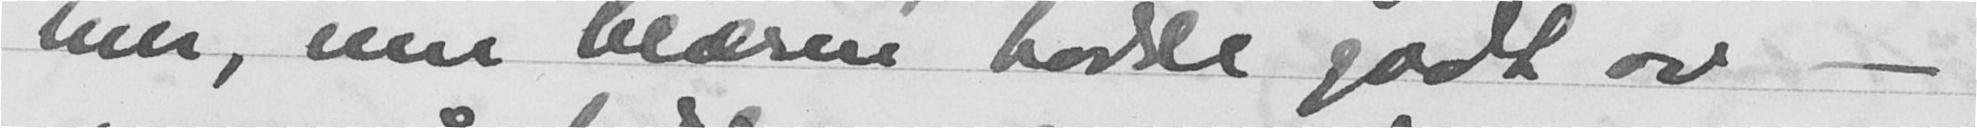mer, enn blæren hadde godt av -
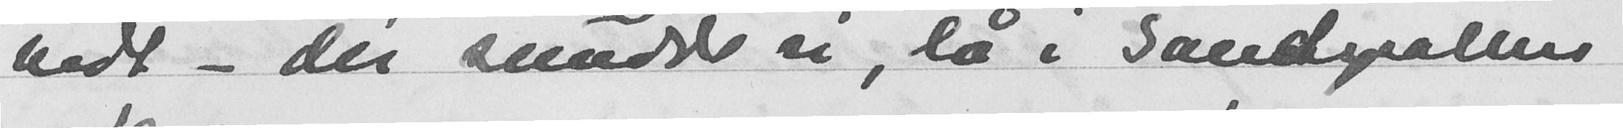vedt - der snudde vi, lå i Sandspollen
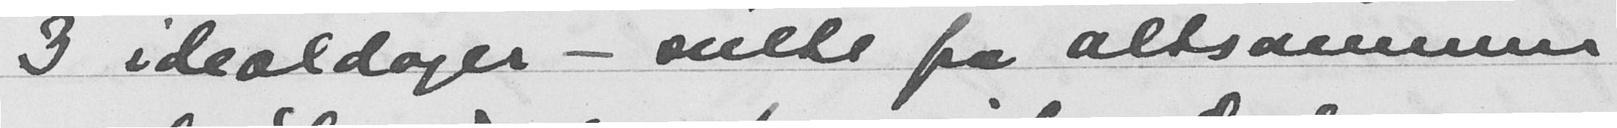3 idealdager - seilte fra altsammen
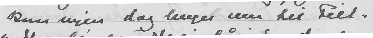kom ingen dag lenger enn til Filt-
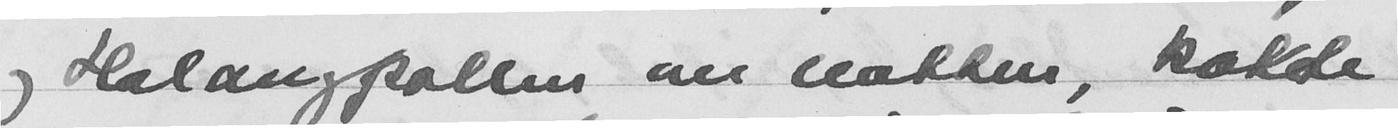og Halangspollen om natten, kokte
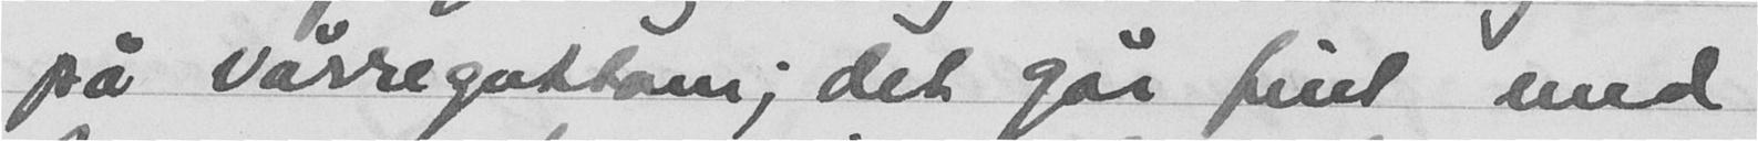på vårregattaen; det går fint med
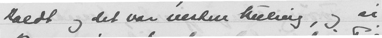kaldt og det var nesten kuling, og vi
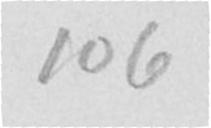106
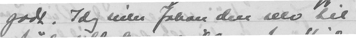godt. Idag seiler Johan den selv til
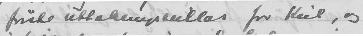første uttakningsseillas for Kiel, og
